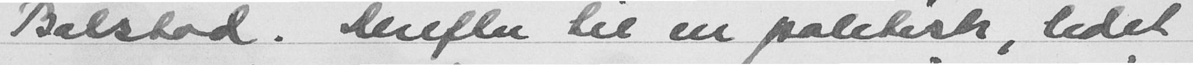Balstad. Derefter til en politisk, ledet
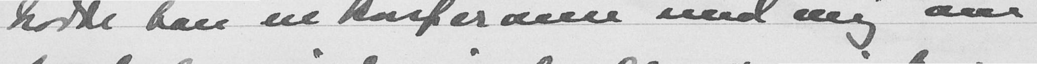hadde han en konferanse med mig om
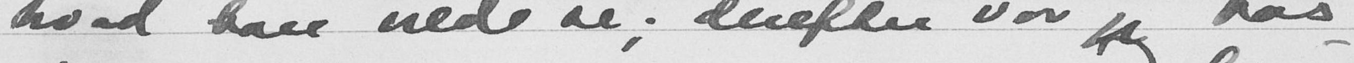hvad han vilde si; derefter var jeg hos
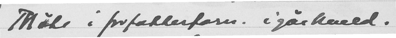Møte i forfatterforn. i gårkveld.
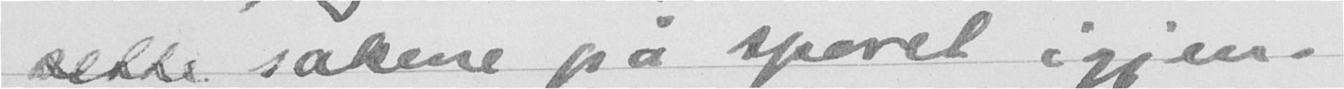sette sakene på sporet igjen.
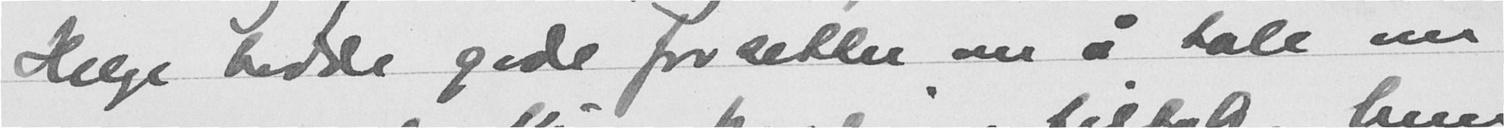Helge hadde gode forsetter om å tale om
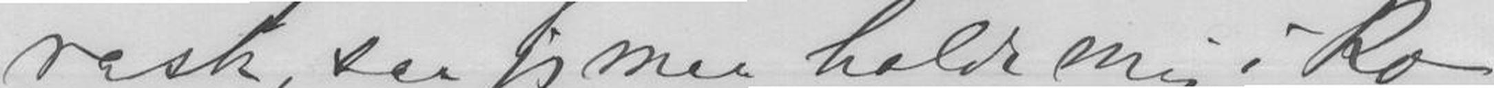rask, saa jeg maa holde mig i Ro
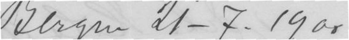Bergen 21-7.1900
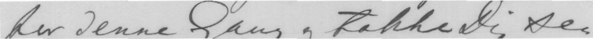for denne Gang og takke Dig saa
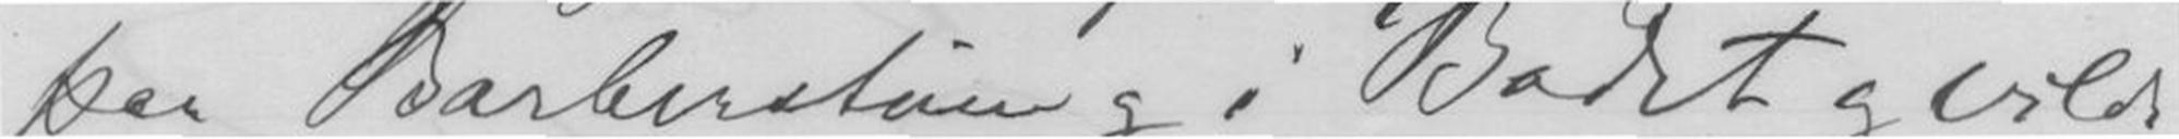paa Barberstuen og i Badet og vilde
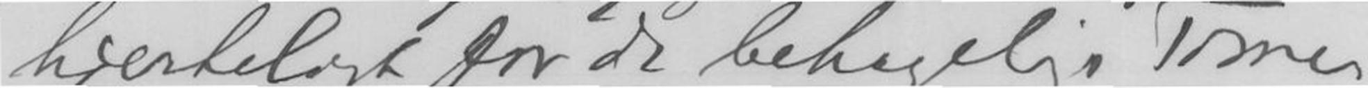hjerteligt for de behagelige Timer
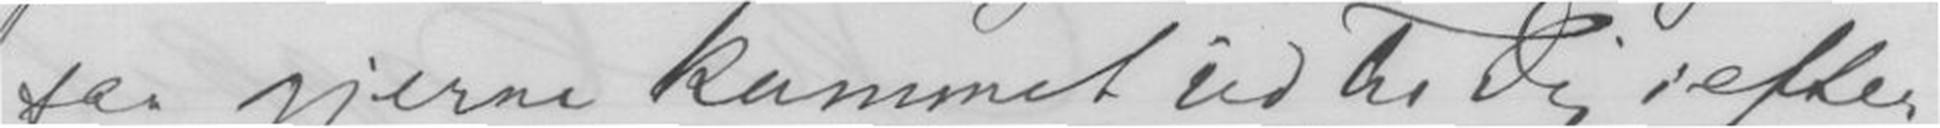saa gjerne kommet ind til Dig iefter-
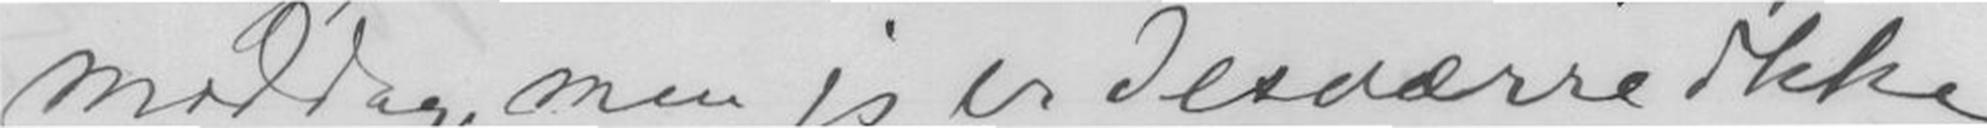middag, men jeg er desværre ikke
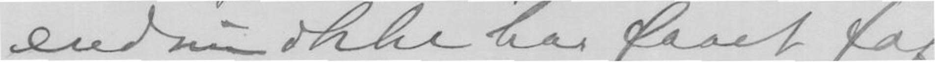endnu ikke har faaet fat
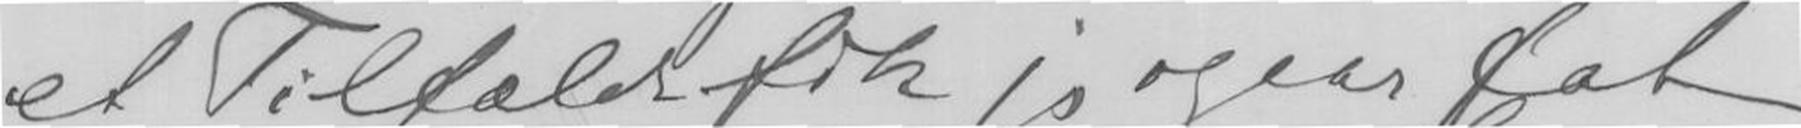et Tilfelde fik jeg ogsaa fat
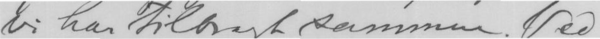vi har tilbragt sammen. Ved
